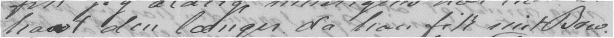havt den læger da han fik mit Brev.
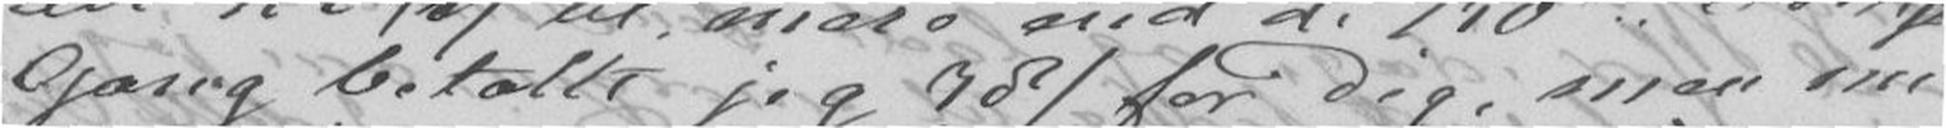Gang betalte jeg 38/ for Dig, men nu
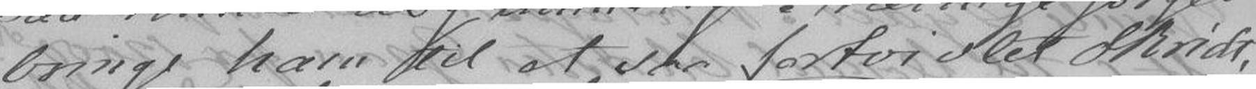bringe ham til et saa fortvivlet Skridt,
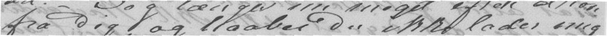fra Dig og haaber Du ikke lader mig
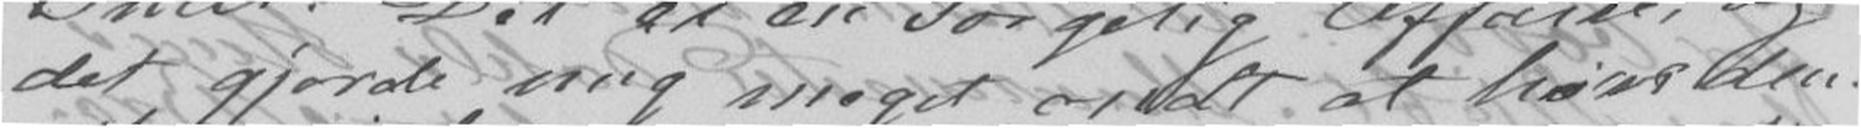det gjorde mig meget ondt at høre den.
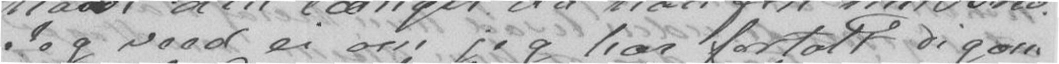Jeg veed ei om jeg har fortalt Dig om
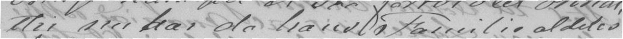thi nu har da hans Familie aldeles
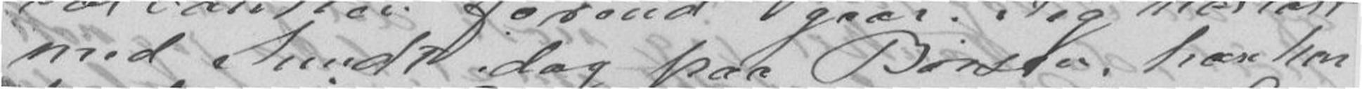med Sundt idag paa Børsen, han har
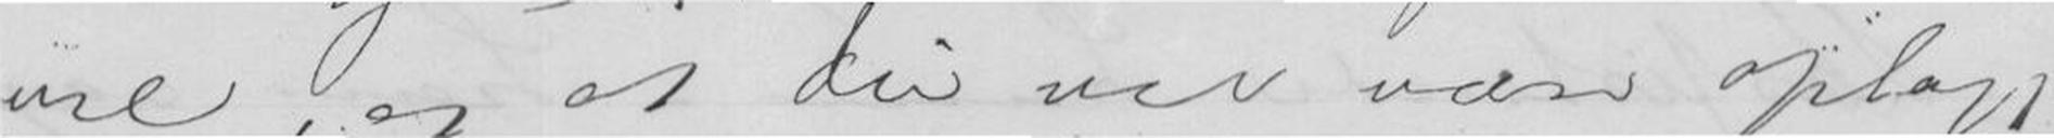vel, og at du vil være oplagt
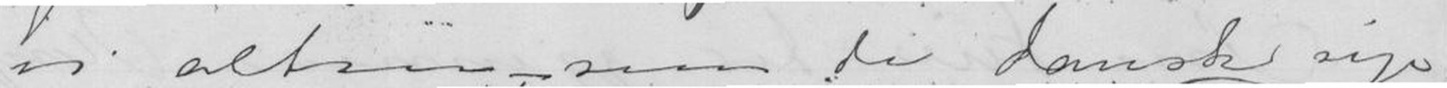vi altsaa - som de danske sige
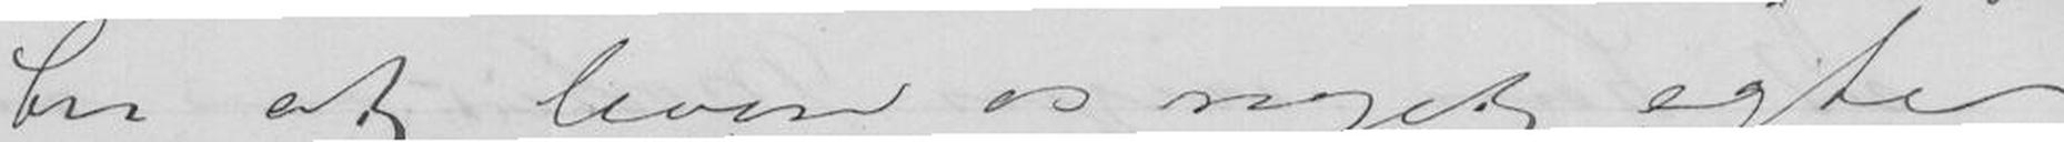til at levere os noget egte
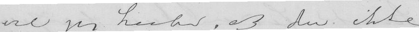vil jeg haabe at Du ikke
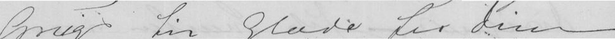Griegs til Glæde for Dine
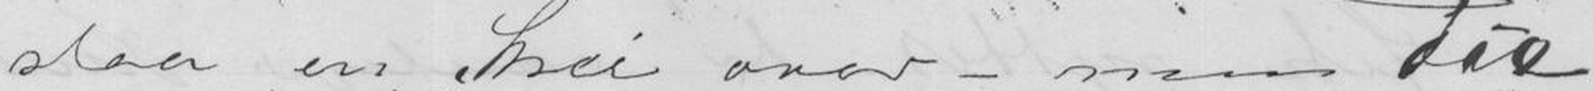slaa en Strec over - men Tid
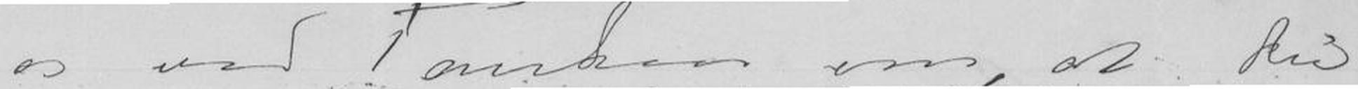os ved Tanken om, at Du
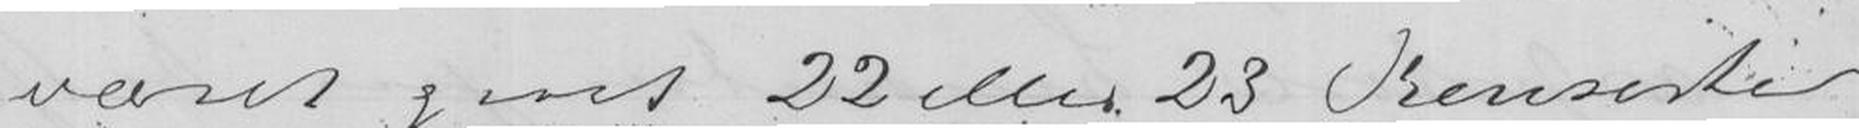været givet 22 ellet 23 Konserter
